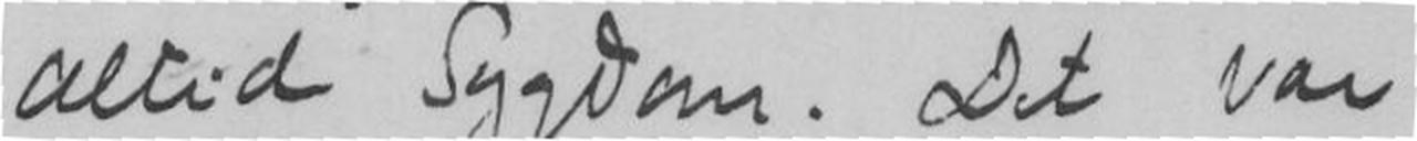altid Sygdom. Det var
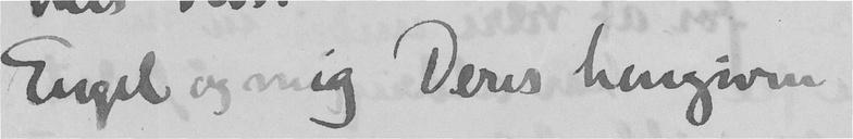Engel og mig Deres hengiven
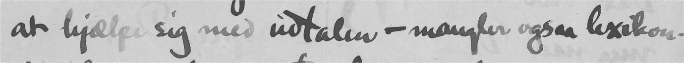at hjælpe sig med udtalen - mangler ogsaa lexikon.
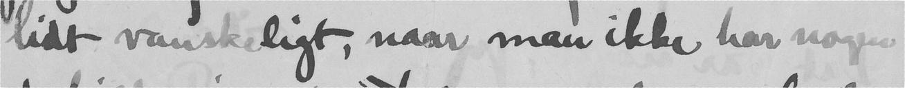lidt vanskeligt, naar man ikke har nogen
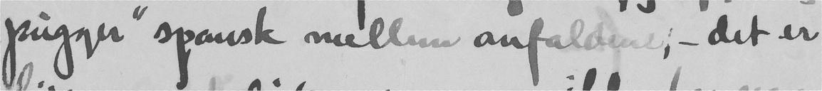"pugger" spansk mellem anfaldene; - det er
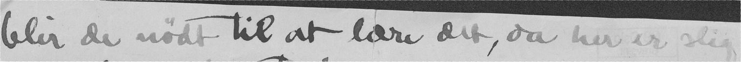blir de nødt til at lære det, da her er slig
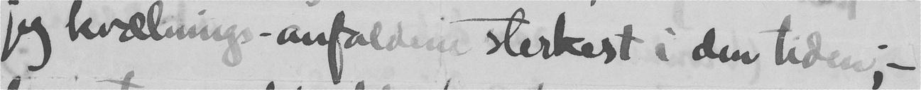jeg kvælning-anfaldene sterkest i den tiden; -
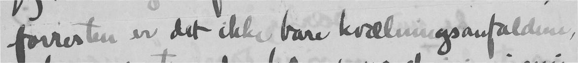forresten er det ikke bare kvælningsanfaldene
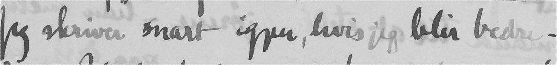Jeg skriver snart igjen, hvis jeg blir bedre.
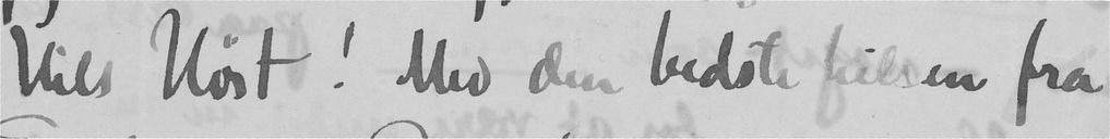Hils Høst! Med den bedste hilsen fra
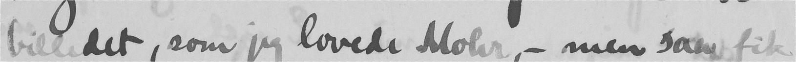biledet, som jeg lovede Mohr, - men som fik
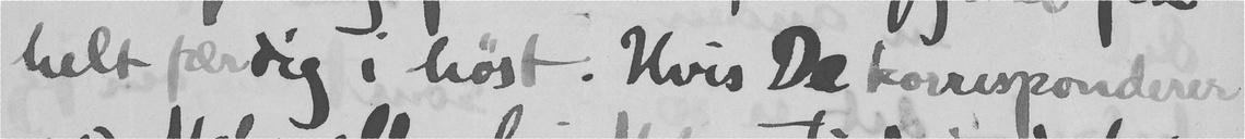helt færdig i høst. Hvis De korresponderer
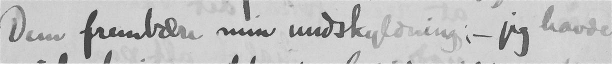Dem frembære min undskyldneng, - jeg havde
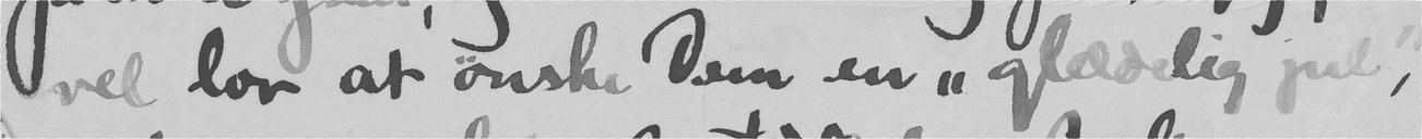vel lov at ønske Dem en "glædelig jul",
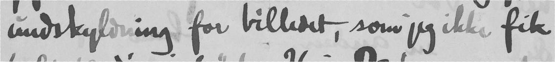undskyldning for billedet, som jeg ikke fik
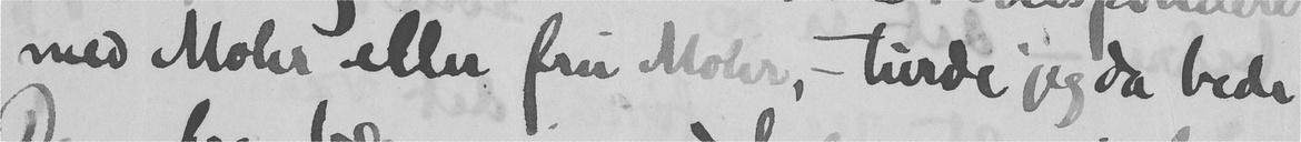med Mohr eller fru Mohr, - turde jeg da bede
